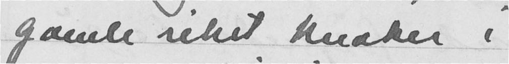gamle riket knaker i
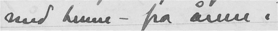med henne - fra årene i
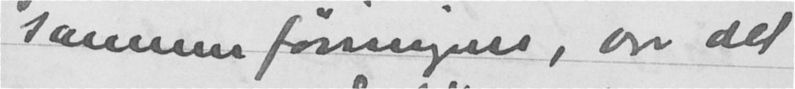sammenføiningene, var det
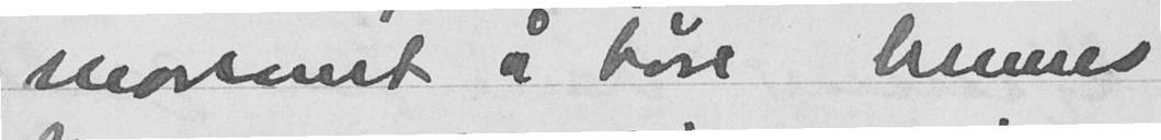morsomt å høre hennes
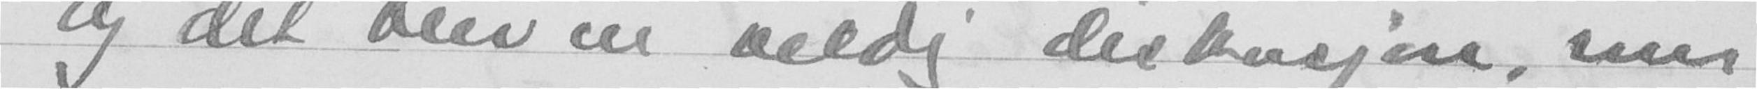Og det blev en veldig diskusjon, men
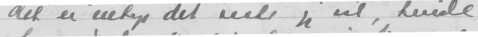det er nettop det siste jeg vil, tende
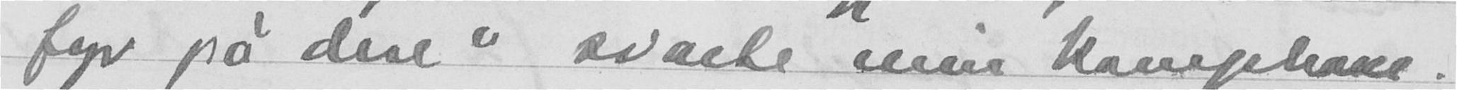fyr på dere" svarte min kamphane.
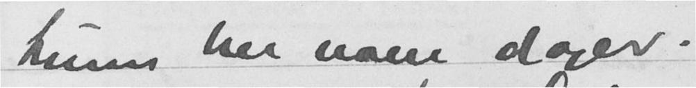Leum her noen dager.
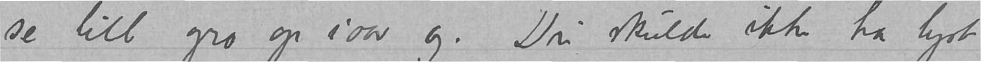see litt gro op iaar og. Du skulde ikke ha lyst
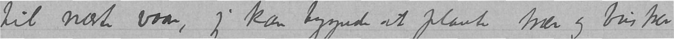til næste vaar, jeg kan begynde at plante trær og busker.
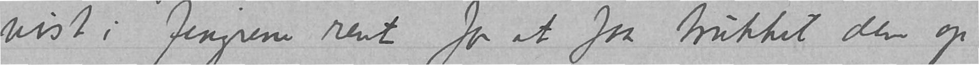vist i fingrene rent for at faa trukket dem op.
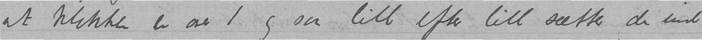at klokken er over 1 og saa litt efter litt sætter de ind,
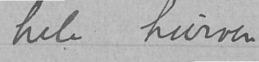hele hurven.
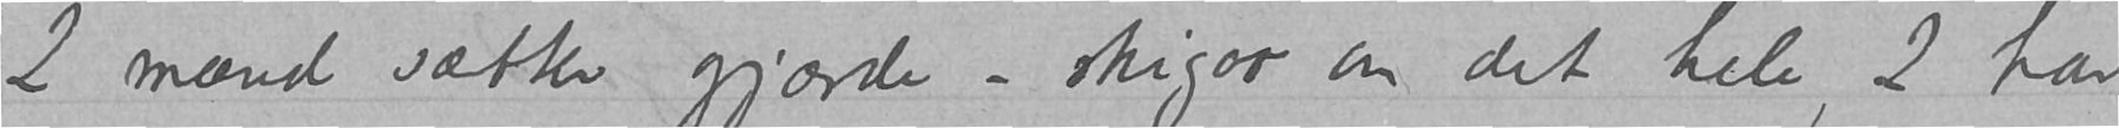2 mænd sætter gjærde –- skigar om det hele, 2 har
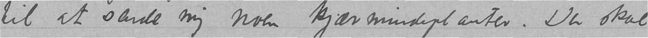til at sænde mig noen kjærmindeplanter. Der skal
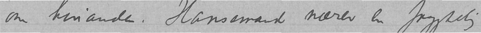om hverandrehinanden.  Hansemand nærer en frygtelig
